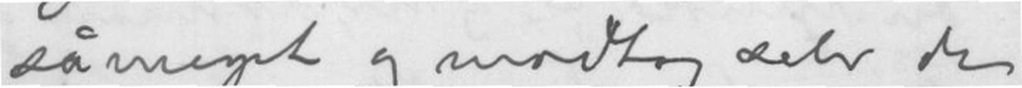så meget og modtag selv de
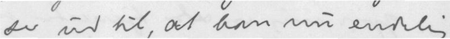ser ud til, at han nu endelig
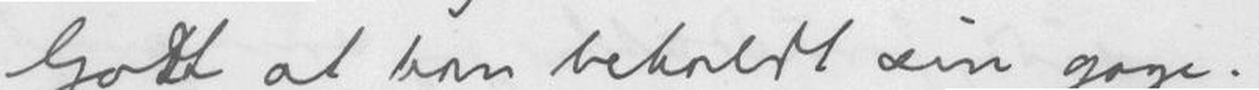Godt at han beholdt sin gage.
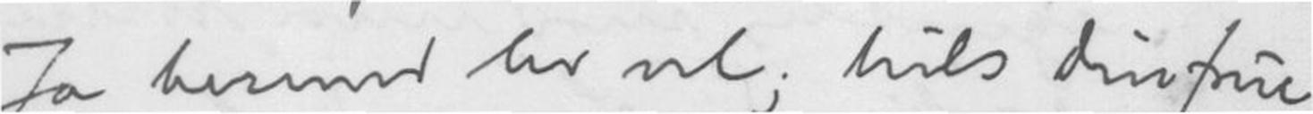Ja hermed lev vel; hils din frue
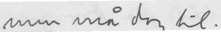men må dog til.-
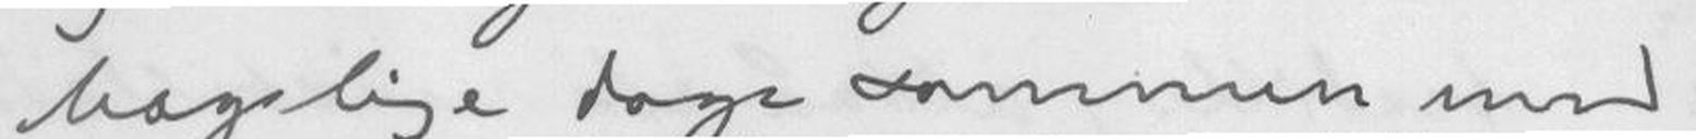hagelige dage sammen med
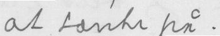at tænke på.
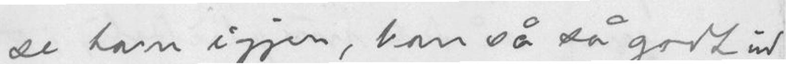se ham igjen, han så så godt ud
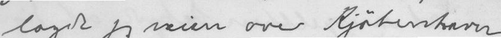lagde jeg veien over Kjøbenhavn
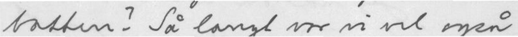batten? Så langt var vi vel også
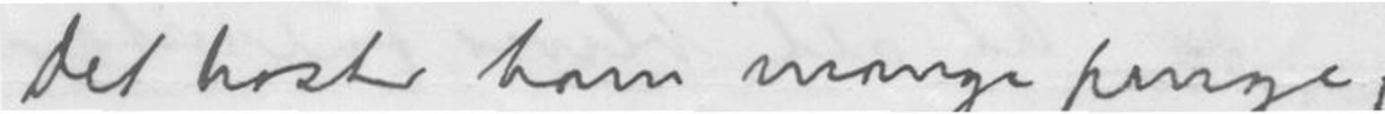Det koster ham mange penge,
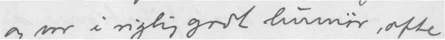og var i rigtig godt humør, ofte
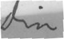din
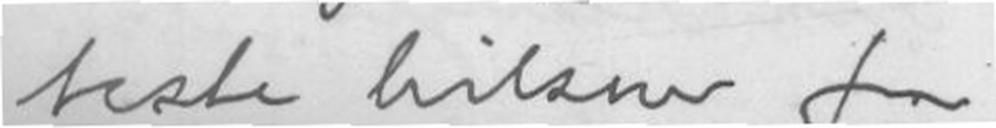bedste hilsner for
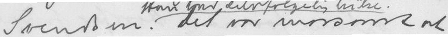Svendsen. det var morsomt at
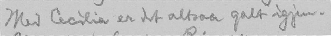Med Cecilia er det altsaa galt igjen.
